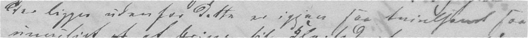der ligger udenfor dette er igjen saa tvivlsomt saa
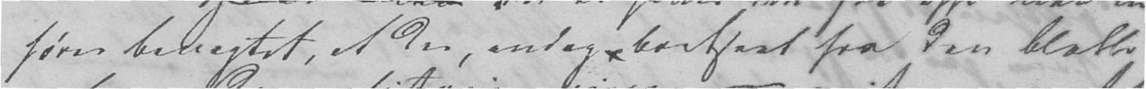hører benægtet, at der, endog bortseet fra den blotte
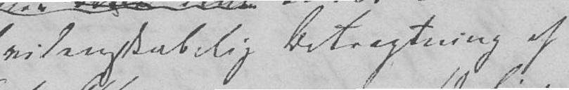videnskabelig Betragtning af
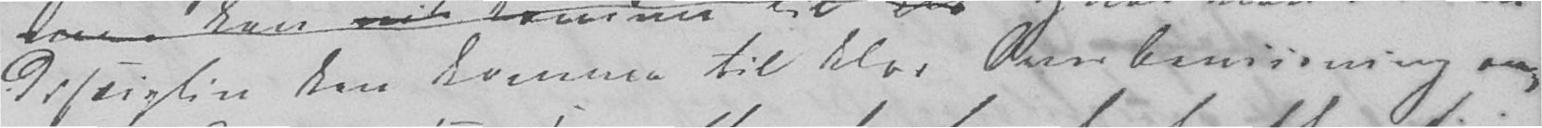Disciplin kan komme til klar Overbeviisning om,
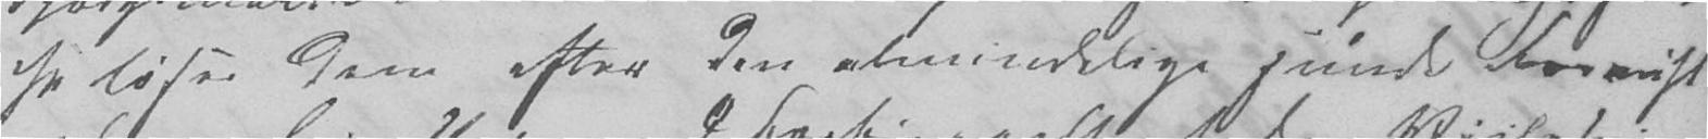se løser dem efter den almindelige sunde Fornuft
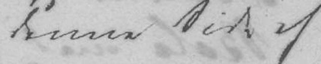denne Side af
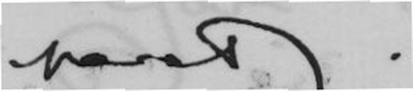parat
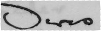Deres
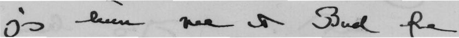jeg kun paa et Bud fra
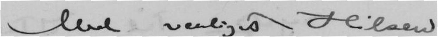Med venligst Hilsen
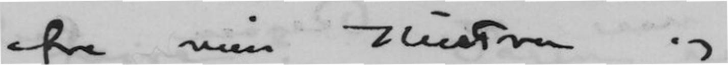fra min Hustru og
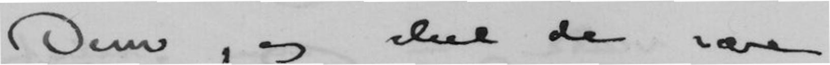Dem, og skal de være
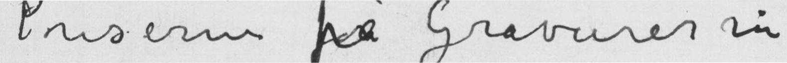Priserne på Gravurerne
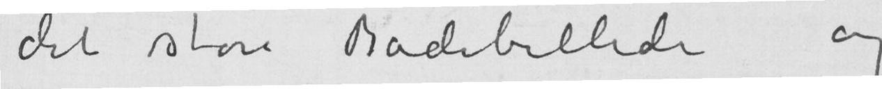det store Badebillede og
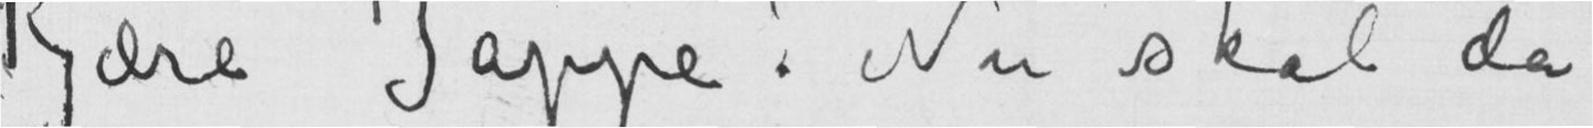Kjære Jappe! Nu skal da
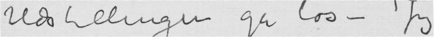Udstillingen gå løs – Jeg
